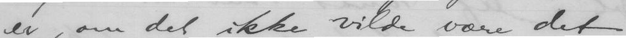er, om det ikke vilde være det
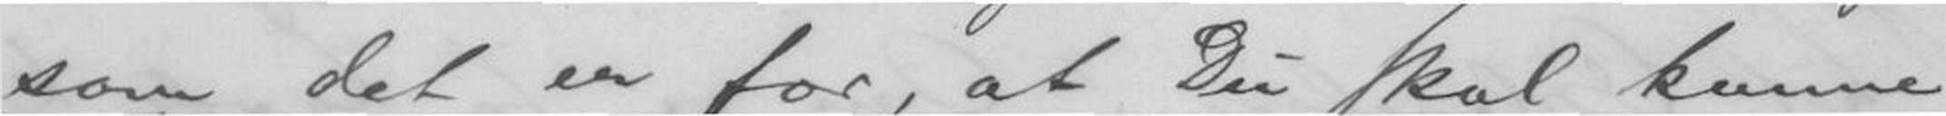som det er for, at Du skal kunne
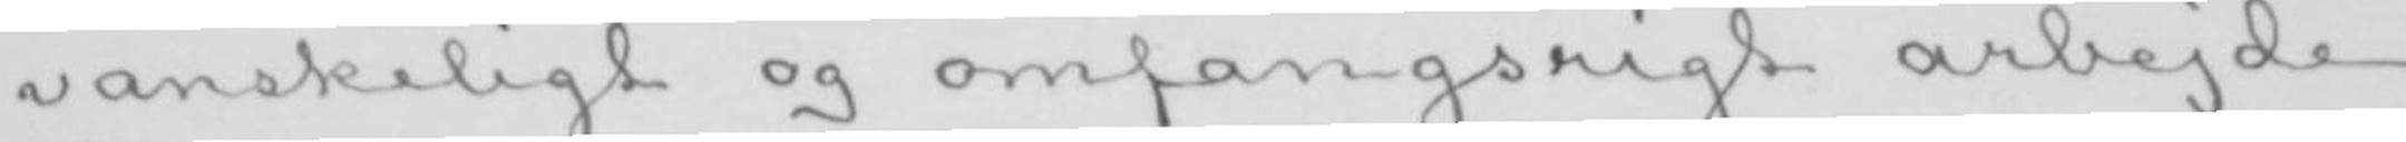vanskeligt og omfangsrigt arbejde.
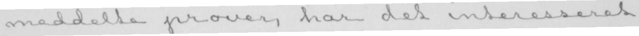meddelte prøver, har det interesseret
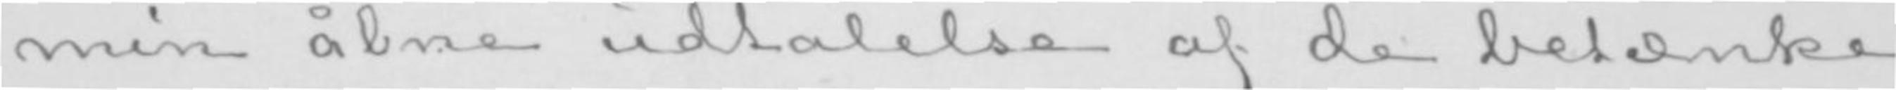min åbne udtalelse af de betænke-
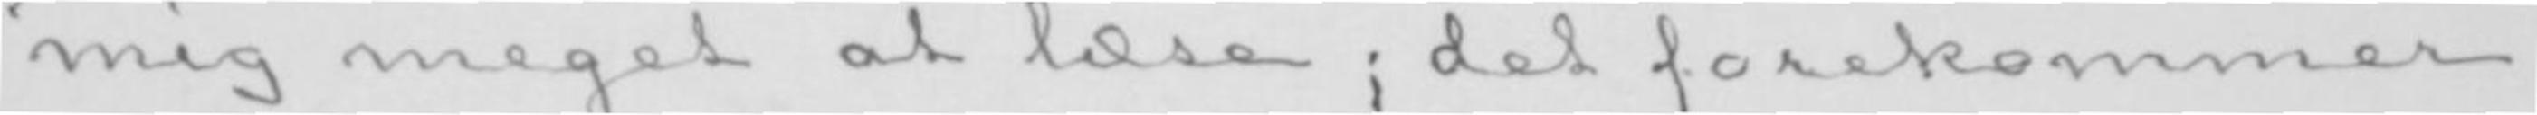mig meget at læse; det forekommer
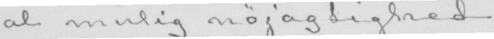al mulig nøjagtighed.
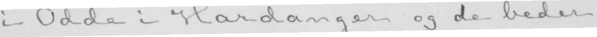i Odde i Hardanger og de beder
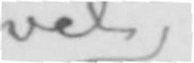vel
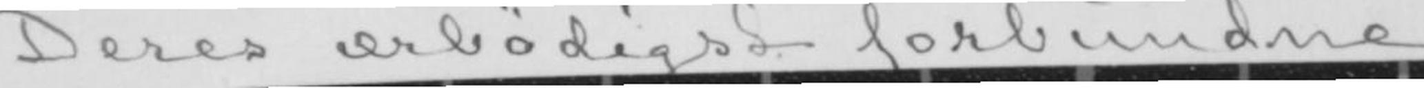Deres ærbødigst forbundne
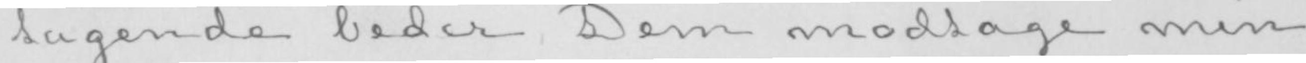tagende beder Dem modtage min
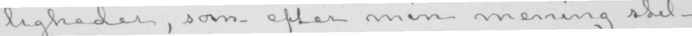ligheder, som efter min mening stil-
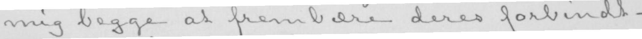mig begge at frembære deres forbindt-
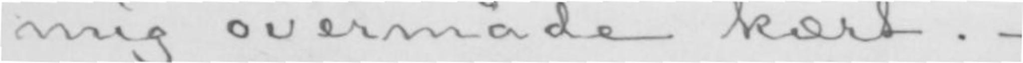mig overmåde kært.-
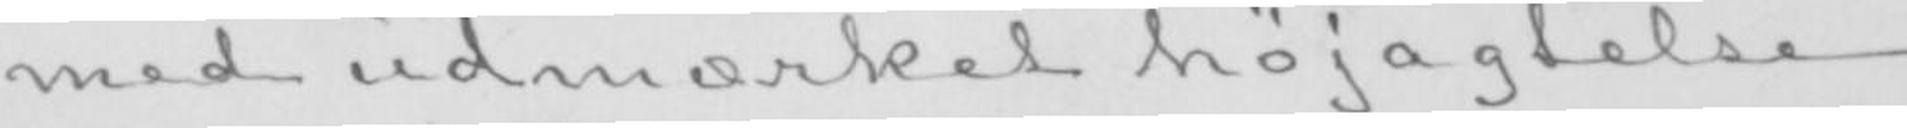med udmærket højagtelse
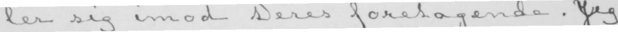ler sig imod Deres foretagende. Jeg
